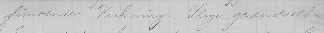glimrende Virkning. Slige grændseløse
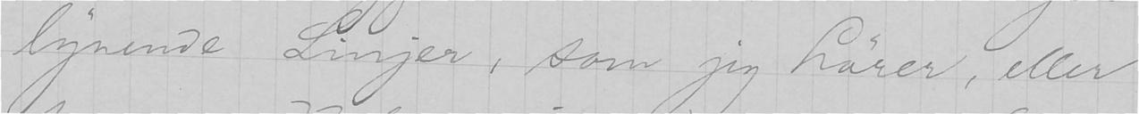lynende Linjer, som jeg hører, eller
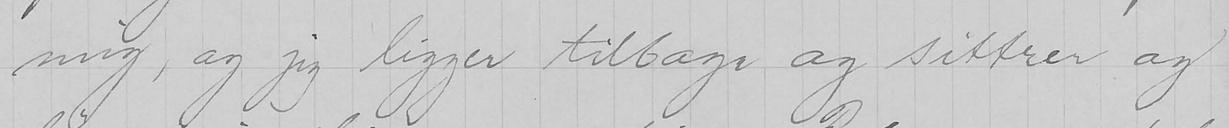mig, og jeg ligger tilbage og sittrer og
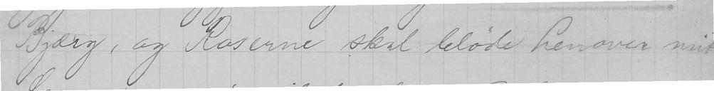Bjærg, og Roserne skal bløde henover mit
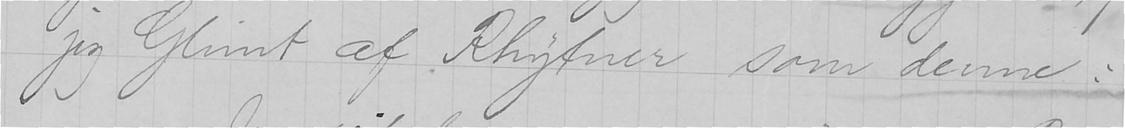jeg Glimt af Rhytmer som denne:
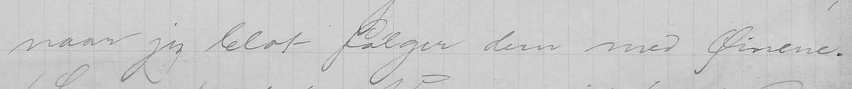naar jeg blot følger dem med Øinene.
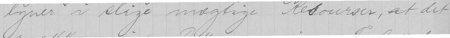lyner i slige mægtige Resourser, at det
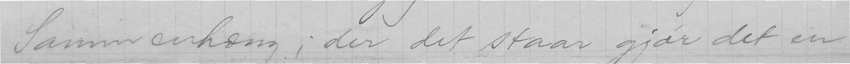Sammenhæng; der det staar gjør det en
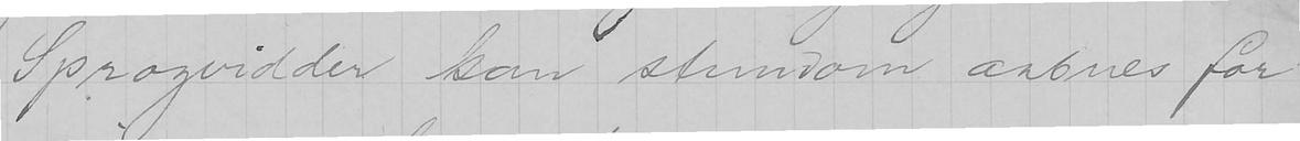Sprogvidder kan stundom aabnes for
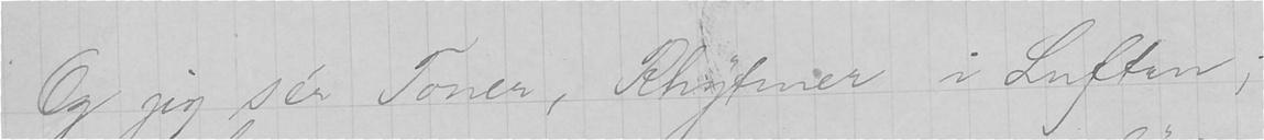Og jeg sér Toner, Rhytmer i Luften;
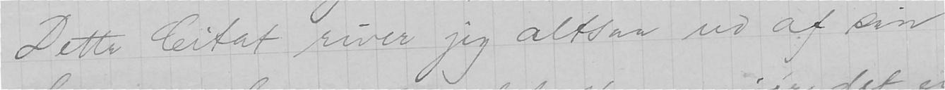Dette Citat river jeg altsaa ud af sin
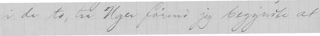i de to, tre Uger førend jeg begyndte at
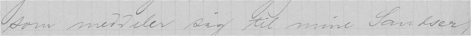som meddeler sig til mine Sandser,
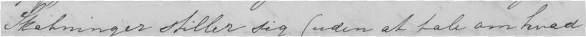Skabninger stiller sig (uden at tale om hvad
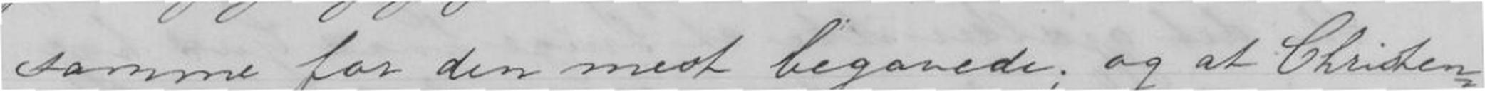samme for den mest begavede; og at Christen-
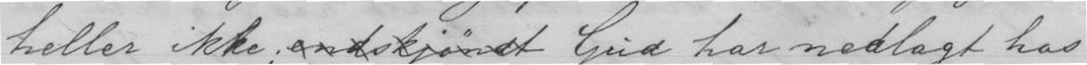heller ikke;endskjøndt Gud har nedlagt hos
